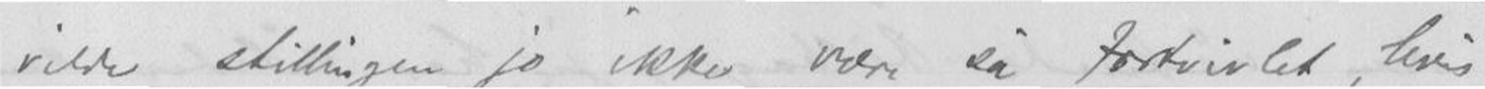vilde stillingen jo ikke være sa fortvilet, hvis
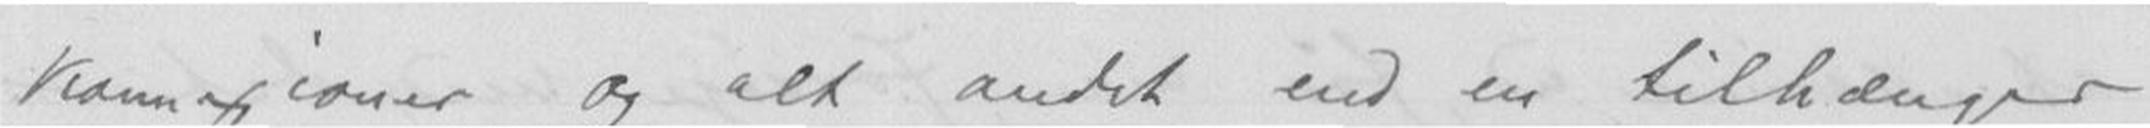komnaxioner? og alt andet end en tilhænger
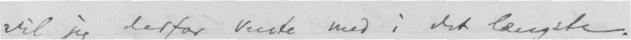vil jeg derfor Vente med i det længste.
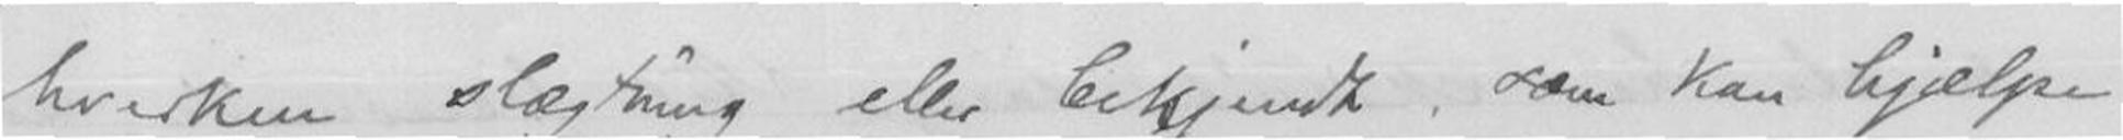hverken slægtning eller bekjendt som kan hjælpe
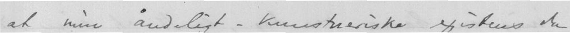at min åndeligt-kunstneriske existens da
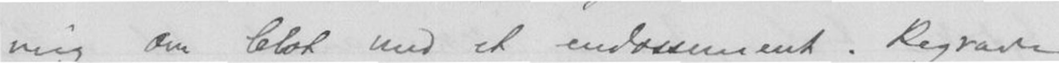mig om blot med et endossement. Begrave
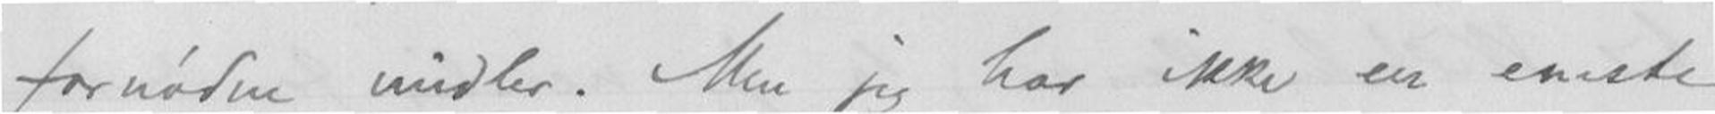fornødne midler. Men jeg har ikke en eneste
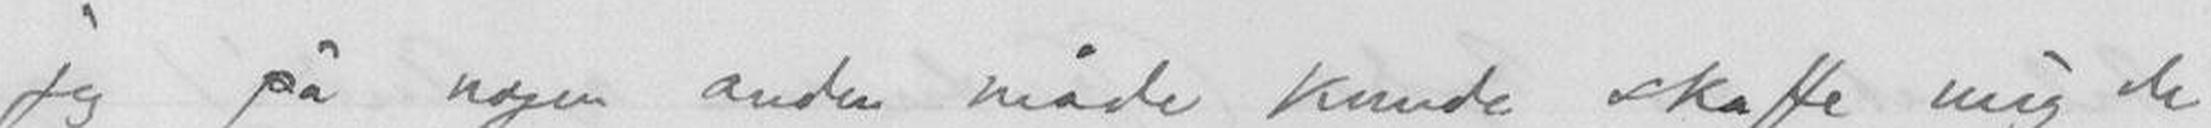jeg på nogen anden måde kunde skaffe mig de
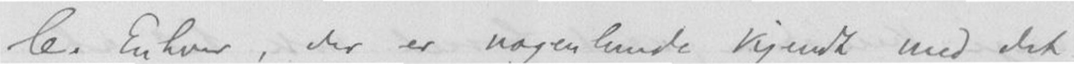le. Enhver, der er nogenlunde kjendte med det
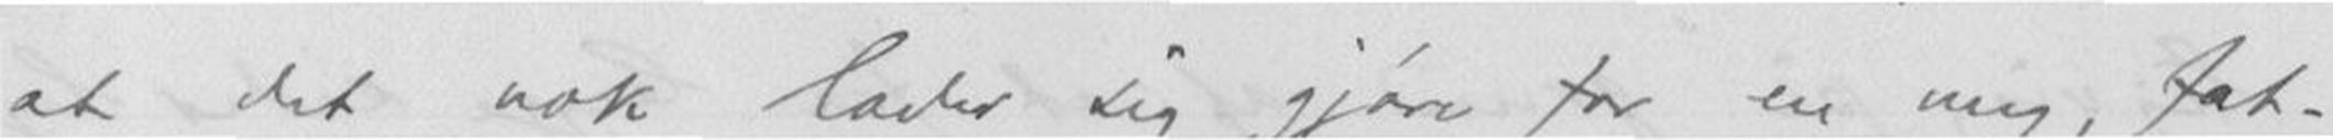at det nok lader sig gjøre for en ung, fat-
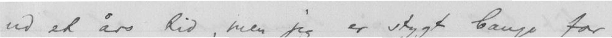ud et års tid, men jeg er stygt bange for.
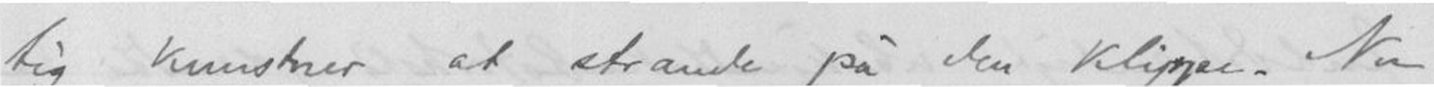tig kunstner at strande på den klippe. Nu
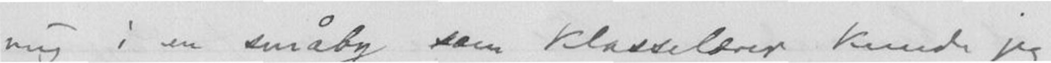mig i en småby som klasselærer kunde jeg
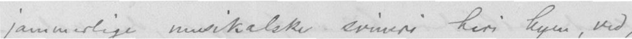jammerlige musikalske svineri heri byen, ved,
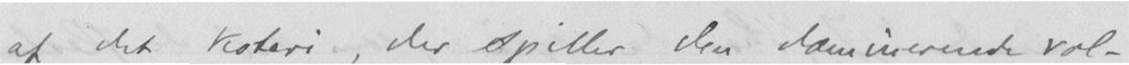af det koteri, der spiller den dominerende rol-
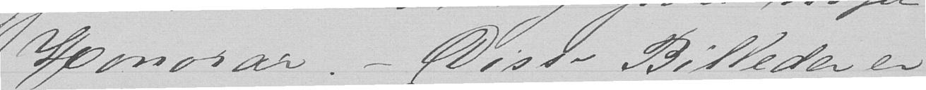Honorar. - Disse Billeder er
